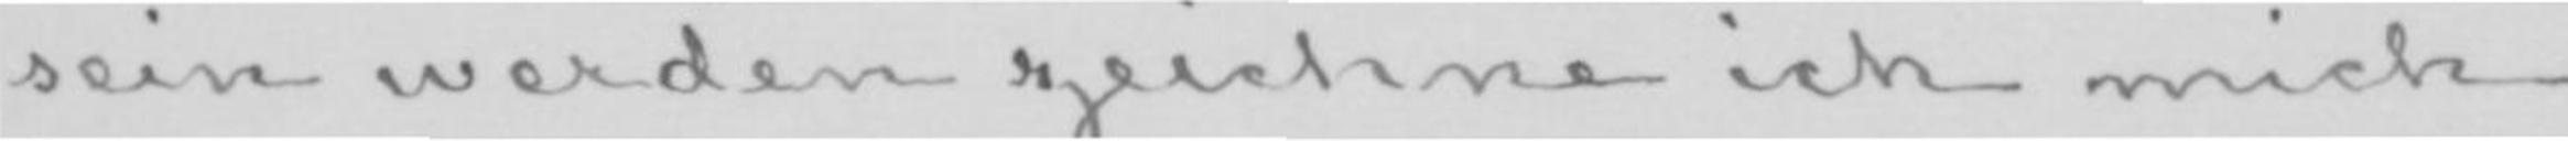sein werden zeichne ich mich
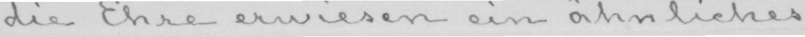die Ehre erwiesen ein ähnliches
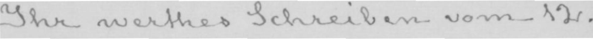Ihr werthes Schreiben vom 12.
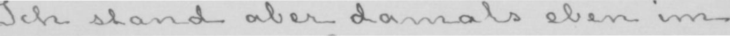Ich stand aber damals eben im
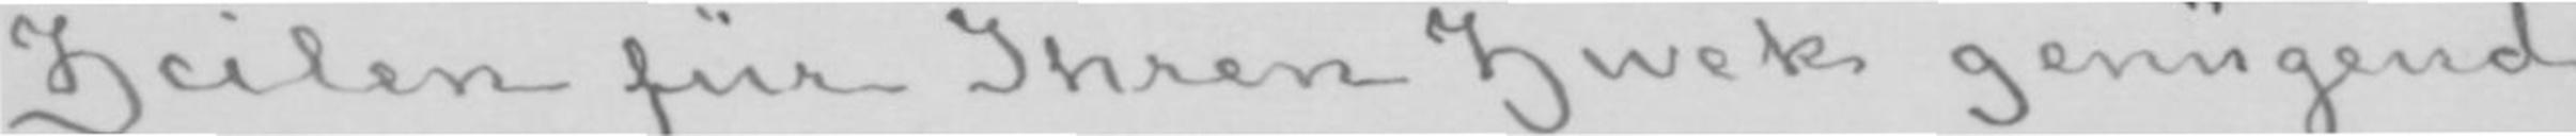Zeilen für Ihren Zwek genügend
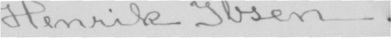Henrik Ibsen.
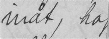mat, har
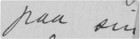paa sin
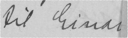til Einar
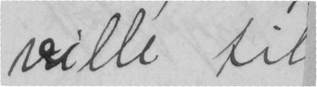ville til
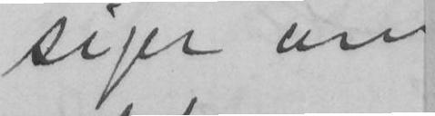siger om
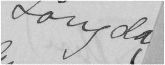sang dag
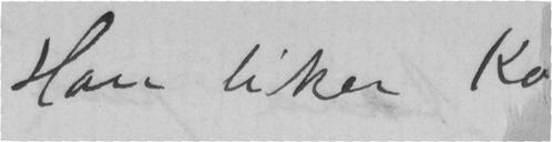Han liker Ka
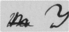saa 3
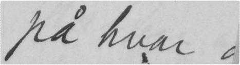pa hvar å
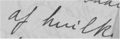af hvilke
